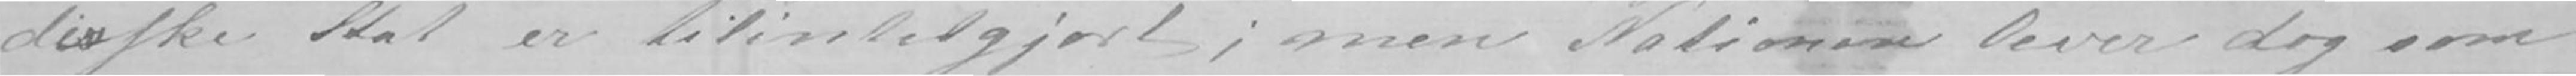diske Stat er tilintetgjort; men Nationen lever dog som
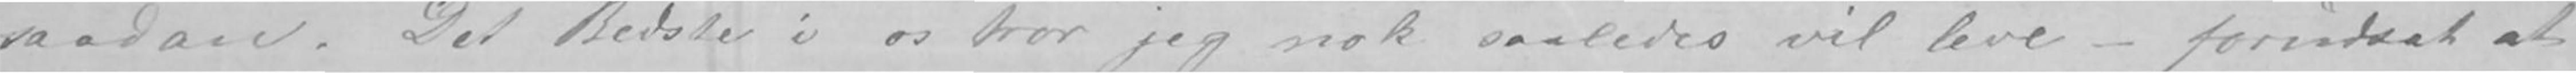saadan. Det Bedste i os tror jeg nok saaledes vil leve- forudsat at
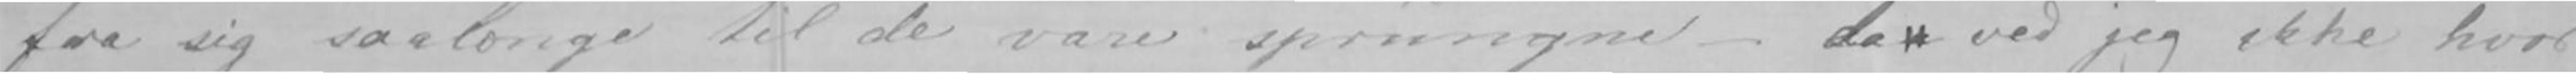fra sig saalænge til de vare sprungne- dasaa ved jeg ikke hvor
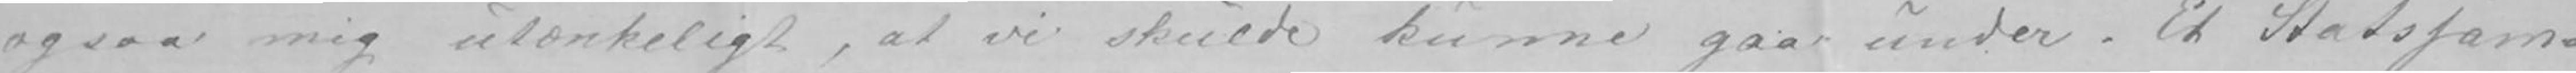ogsaa mig utænkeligt, at vi skulde kunne gaa under. Et Statssam-
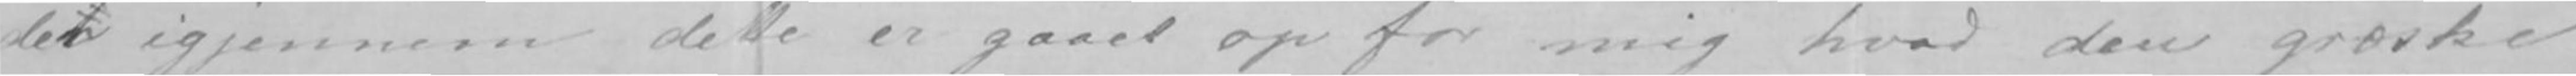derdet igjennem dette er gaaet op for mig hvad den græske
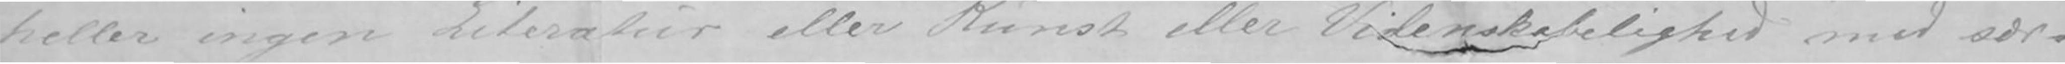heller ingen Literatur eller Kunst eller Videnskabelighed med sær-
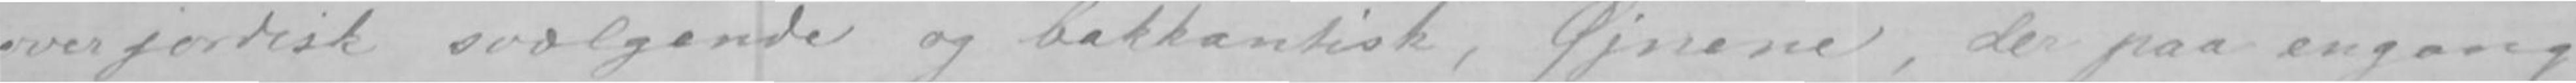overjordisk svælgende og bakkantisk, Øjnene, der paa engang
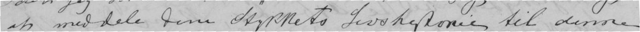at meddele Dem Stykkets Livshistorie til denne
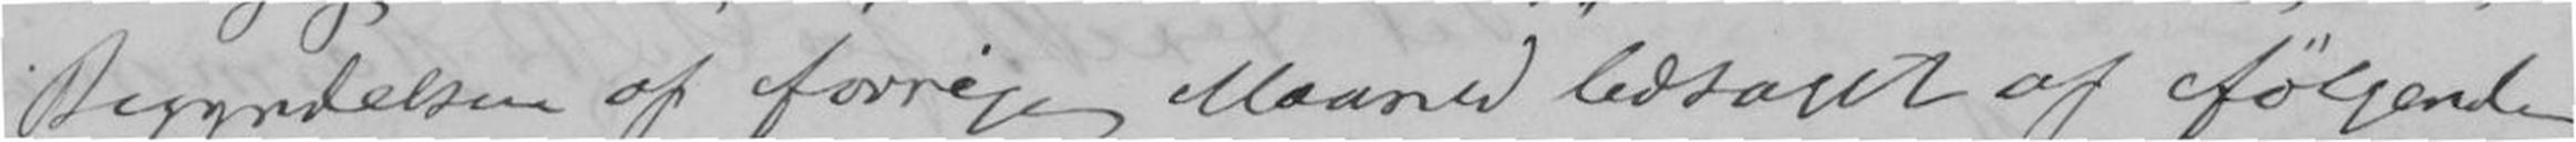Begyndelsen af forige Maaned ledsaget af følgende
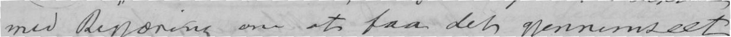med Begjæring om at faa det gjennemseet
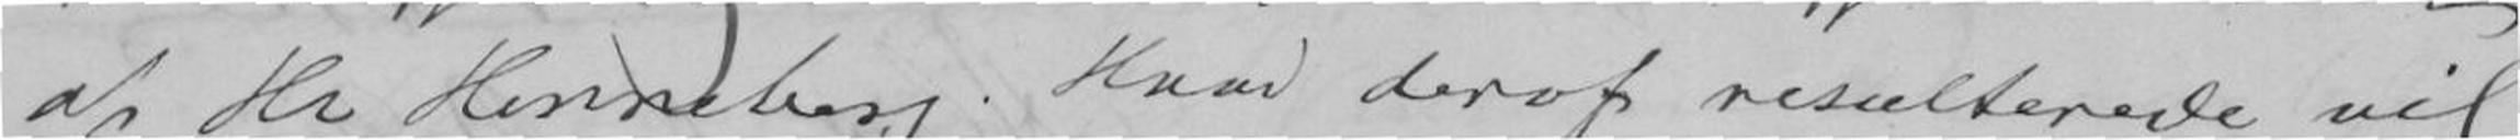af Hr Henneberg. Hvad deraf resulterede vil
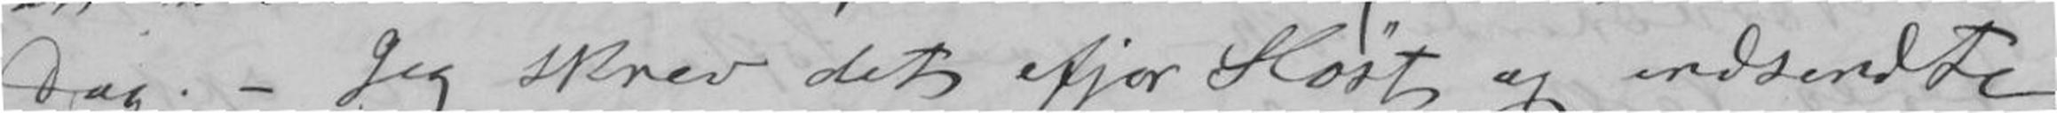Dag. - Jeg skrev det ifjor Høst og indsendte
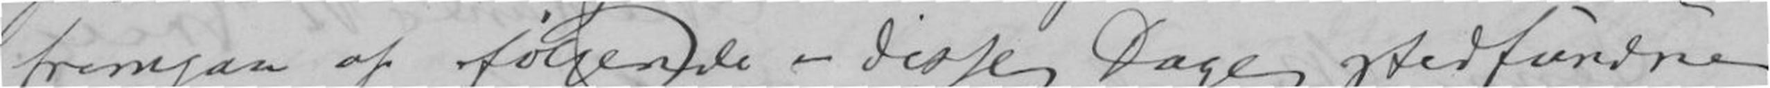fremgaa af følgende - disse Dage stedfundne
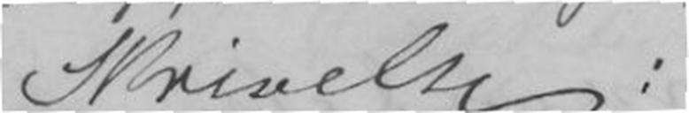Skrivelse:
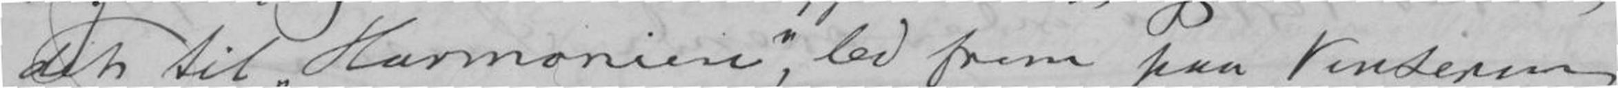det til Harmonien, led frem paa Vinteren
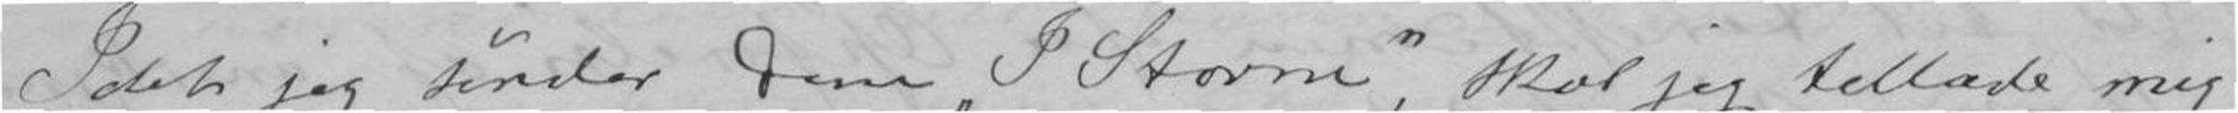Idet jeg sender Dem I Storm, skal jeg tillade mig
